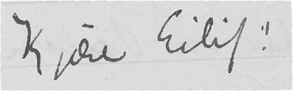Kjære Eilif!
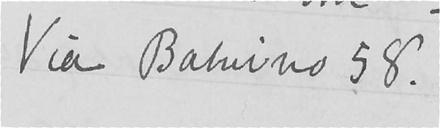Via Babuino 58.
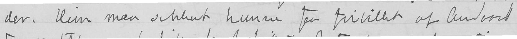der. Hun maa sikkert kunne faa fribillet af Andvord
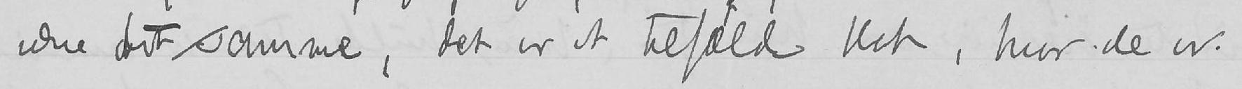være det samme, det er et tilfælde blot, hvor de er.
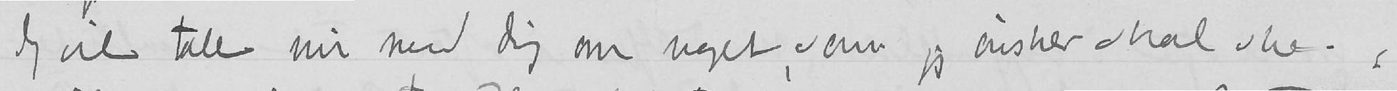Jeg vil tale nu med dig om noget, som jeg ønsker skal ske –,
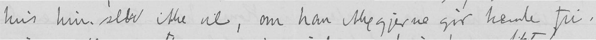hvis hun selv ikke vil, om han ikke gjerne gir hende fri-
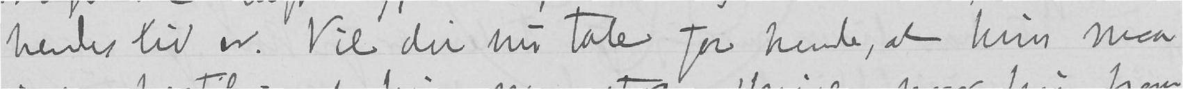hendes liv er. Vil du nu tale for hende, at hun maa
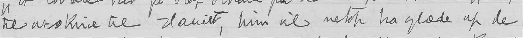til at skrive til Harriet, hun vil netop ha glæde af de
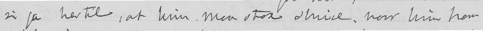si ja hertil, at hun maa strax skrive, naar hun kan
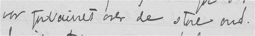var forbauset over de store ord.
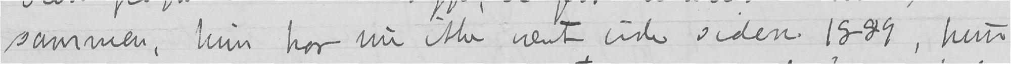sammen, hun har nu ikke vært ude siden 1889, hun
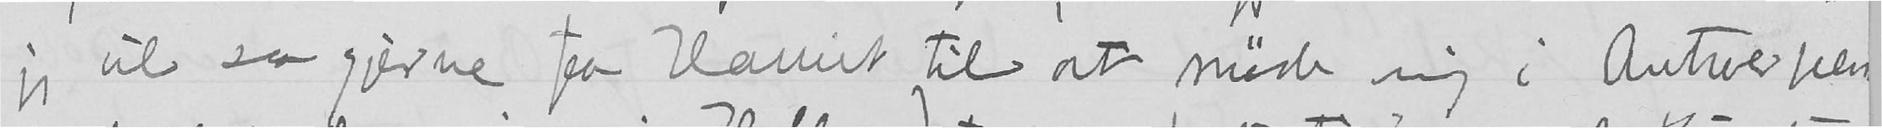jeg vil saa gjerne faa Harriet til at møde mig i Antwerpen,
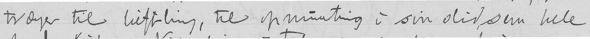trænger til luftling, til opmuntring i sin slid, som hele
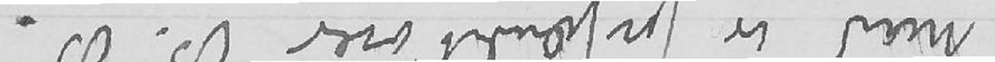med er forfærdet over B. B.
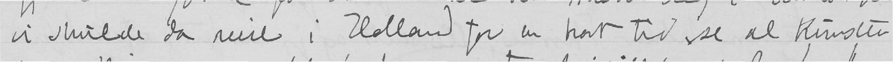vi skulde da reise i Holland for en kort tid, se al kunsten
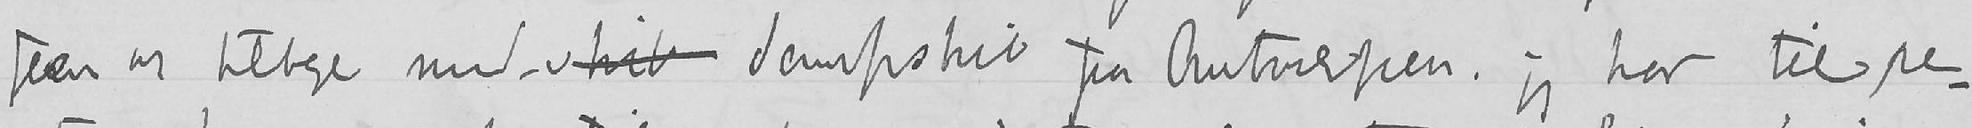frem og tilbage med skib dampskib fra Antwerpen, jeg har til re-
